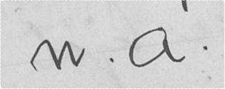n.a.
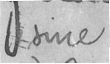sine
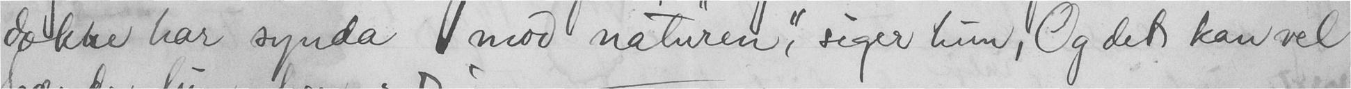dokke har synda mod naturen," siger hun. Og det kan vel
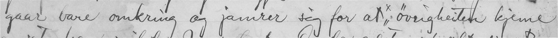gaar bare omkring og jamrer sig for at "øvrigheiten kjeme
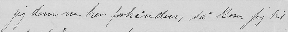jeg dem nu her forhånden, så kom jeg til
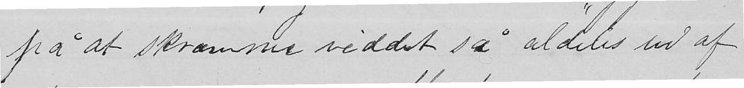på at skræmme viddet så aldeles ud af
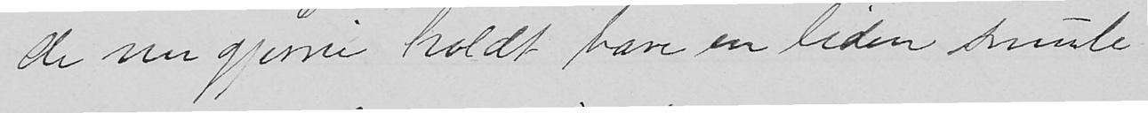de nu gjerne holdt bare en liden smule
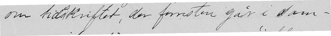om tidskriftet, der forresten går i sam-
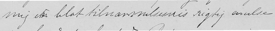mig en blot tilnærmelsessvis rigtig anelse
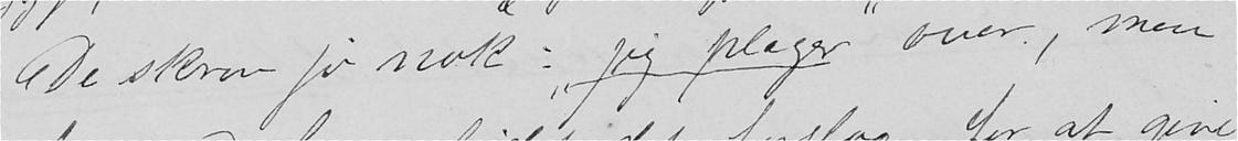De skrev jo nok: "jeg plager" over, men
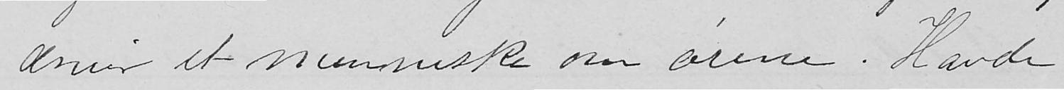dirrer et menneske om ørene. Havde
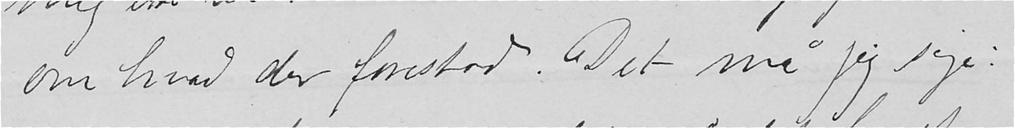om hvad der forestod. Det må jeg sige:
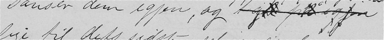sanser dem igjen, og gik på igjen
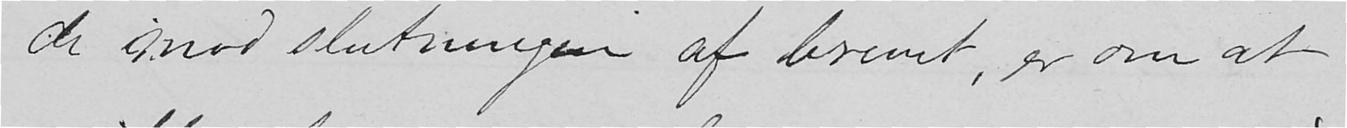de mod slutningen af brevet, er om at
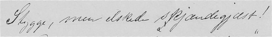Stygge, men elskede skjændegjæst!
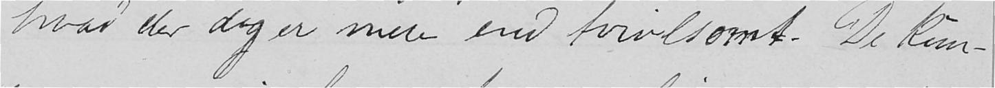hvad der dog er mere end tvivlsomt. De kun-
